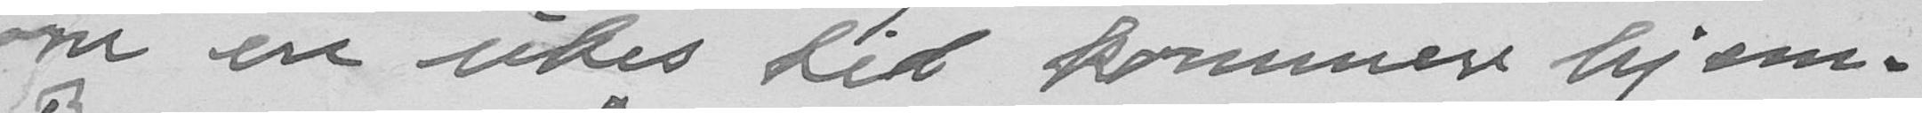om en ukes tid kommer hjem.
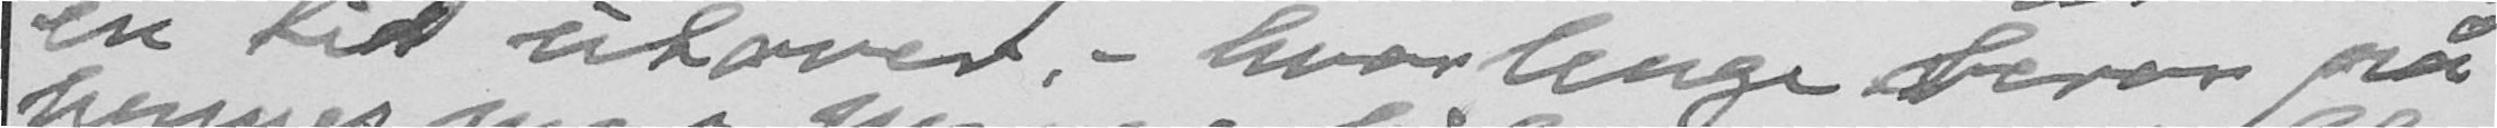en tid utover, - hvor lenge beror på
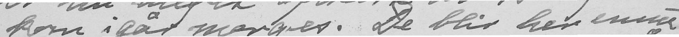kom i går morges. De blir her ennu
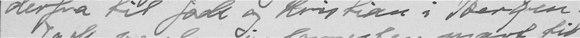derfra til Jack og Kristian i Bergen.
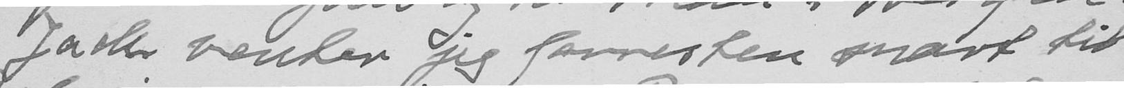Jack venter jeg forresten snart til
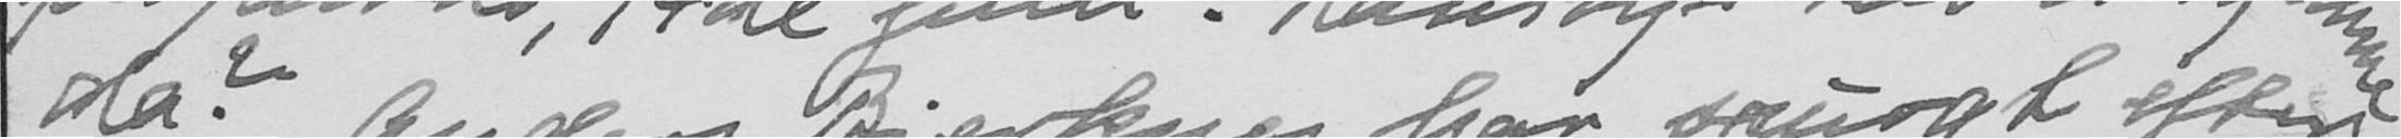da? Anders Bjerknes har spurgt efter
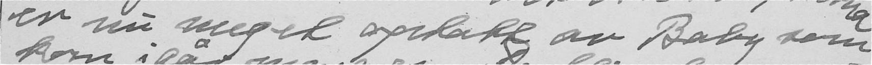er nu meget optatt av Baby som
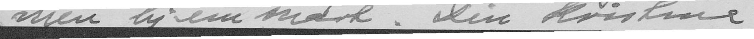men hjem snart. Din Kristine
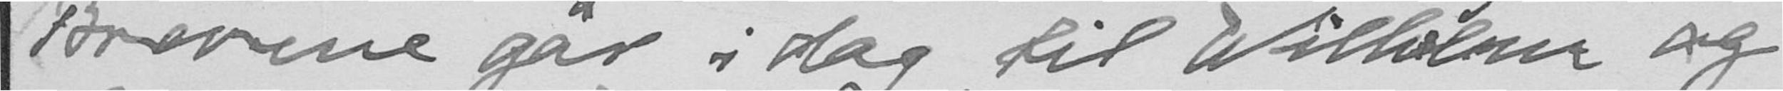Brevene går i dag til Wilhelm og
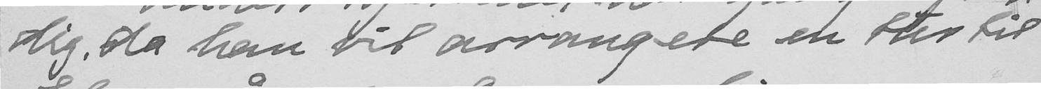dig, da han vil arrangere en tur til
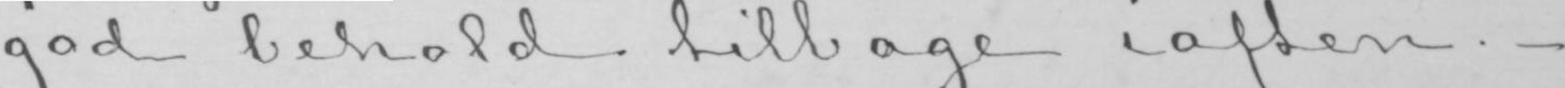god behold tilbage iaften.-
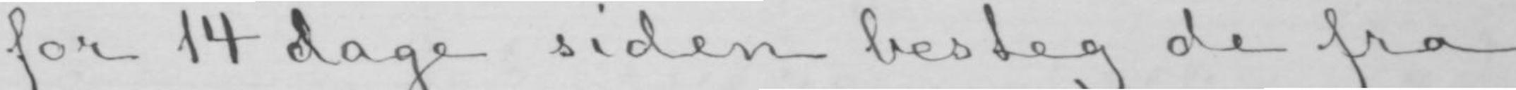for 14 dage siden besteg de fra
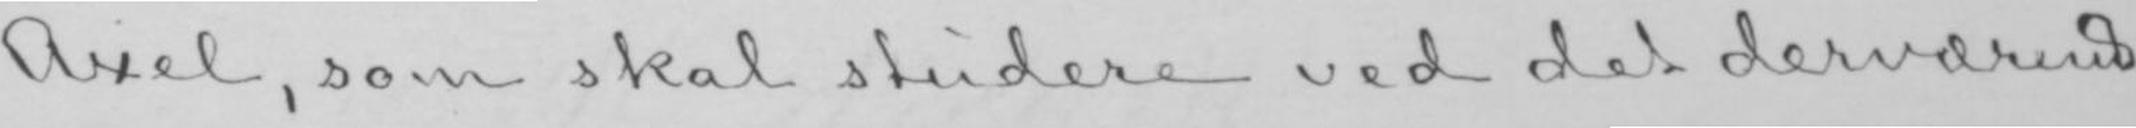Axel, som skal studere ved det derværende
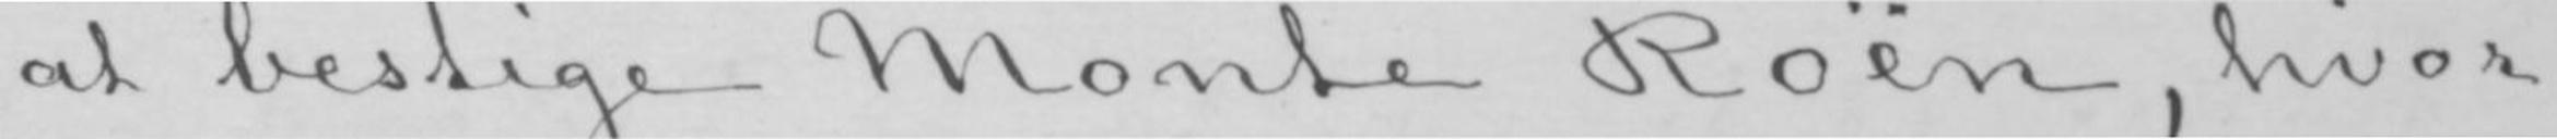at bestige Monte Roën, hvor-
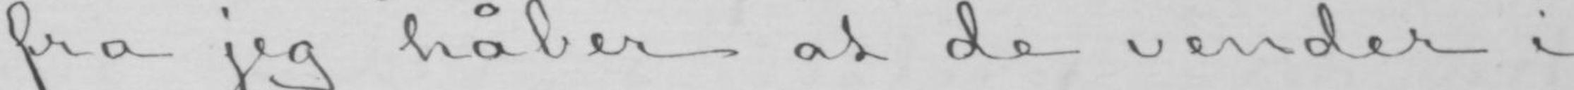fra jeg håber at de vender i
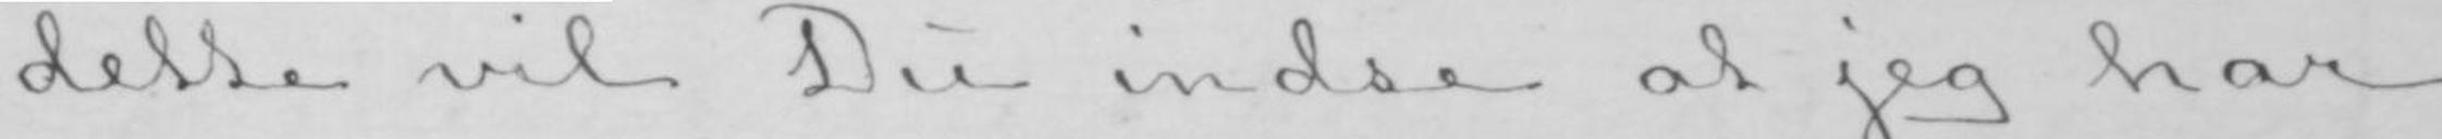dette vil Du indse at jeg har
